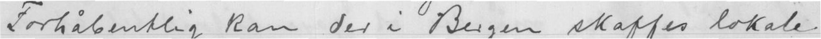Forhåbentlig kan der i Bergen skaffes lokale,
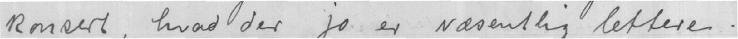konsert, hvad der jo er væsentlig lettere.
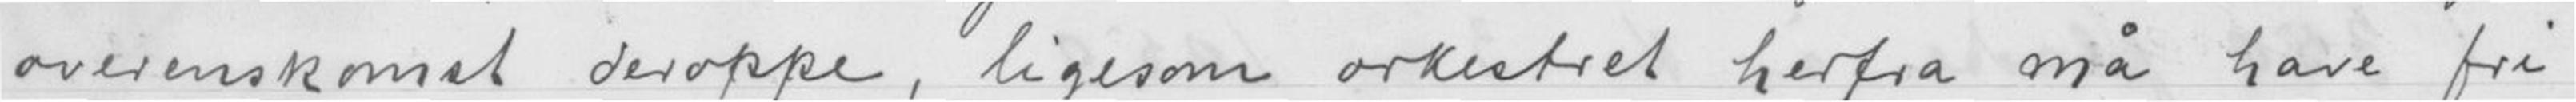overenskomst deroppe, ligesom orkestret herfra må have fri
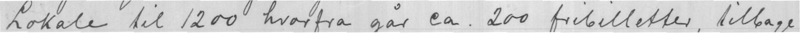Lokale til 1200 hvorfra går ca.200 fribilletter,tilbage
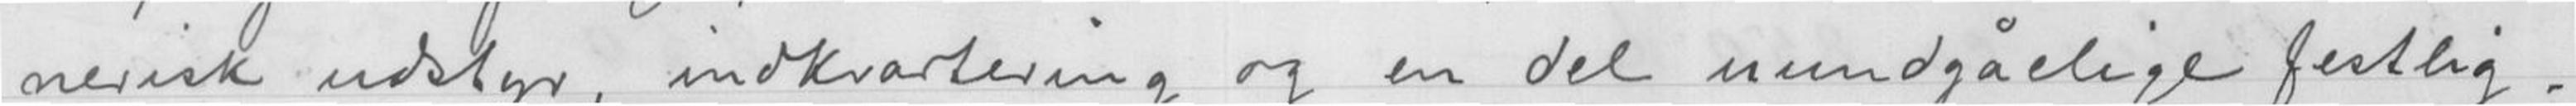nerisk udstyr, indkvatering og en del uundgåelige festlig-
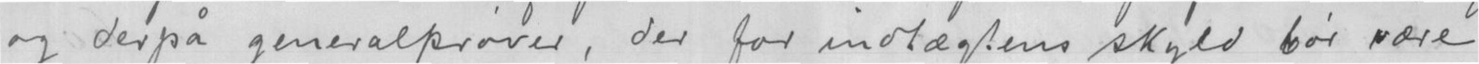og derpå generalprøver, der for indtægtens skyld bør være
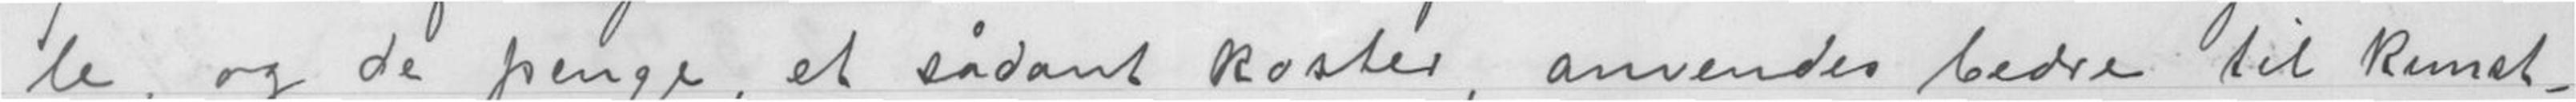le, og de penge, et sådant koster, anvendes bedre til Kunst-
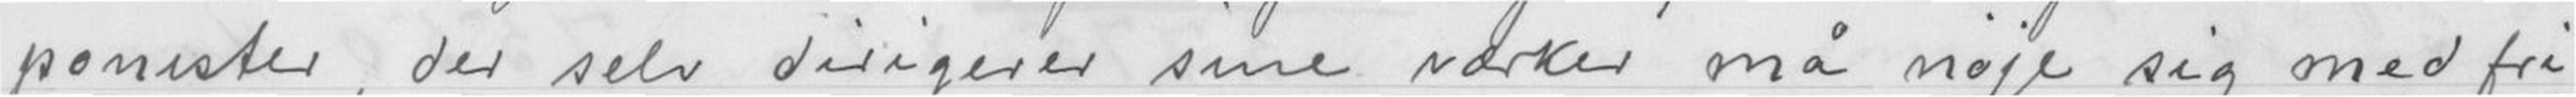ponister, der selv dirigerer sine værker må nøje sig med fri
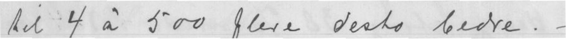til 4 à 500 flere desto bedre.-
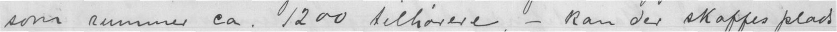som rummer ca. 1200 tilhørere,- kan der skaffes plads
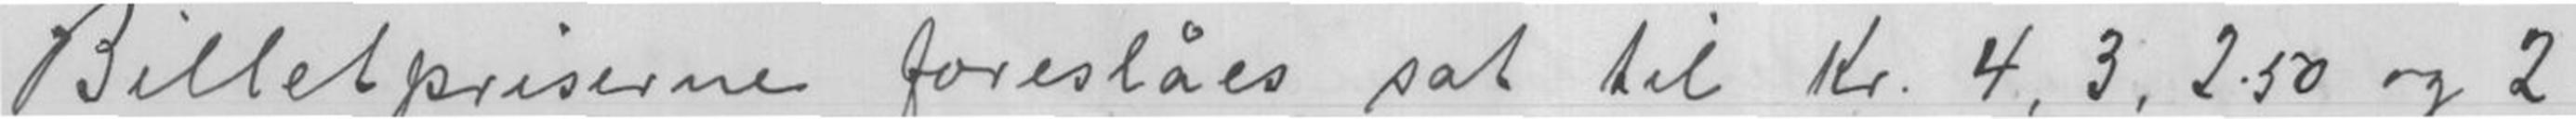Billetprisene foreslåes sat til Kr. 4, 3, 2.50 og 2
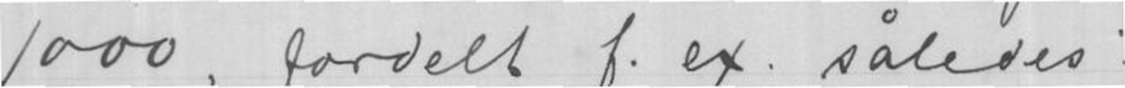1000, fordelt f.ex. således:
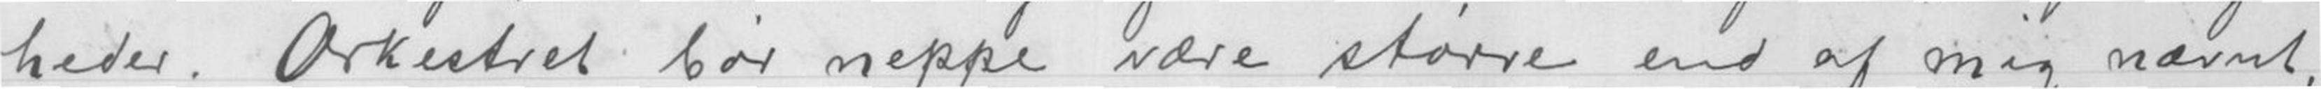heder. Orkestret bør neppe være større end af mig nævnt,
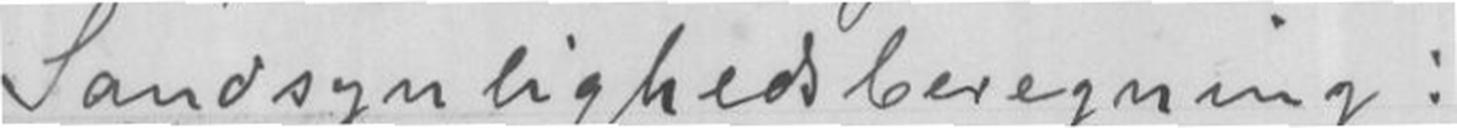Sandsynlighedsberegning.
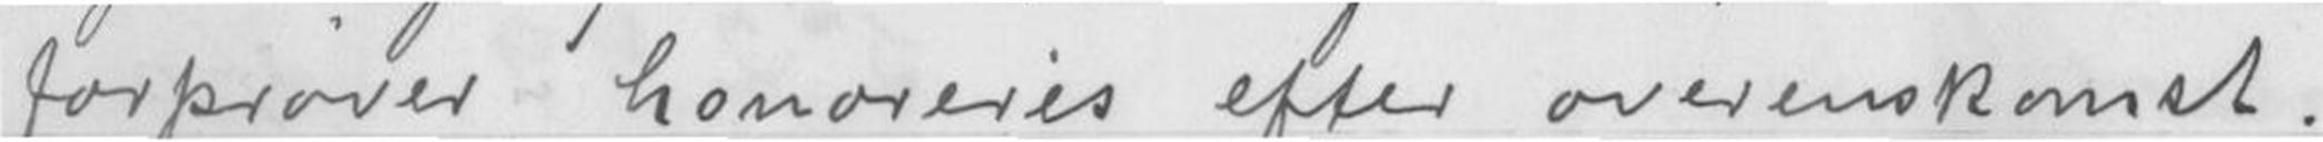forprøver, honoreres efter overenskomst.
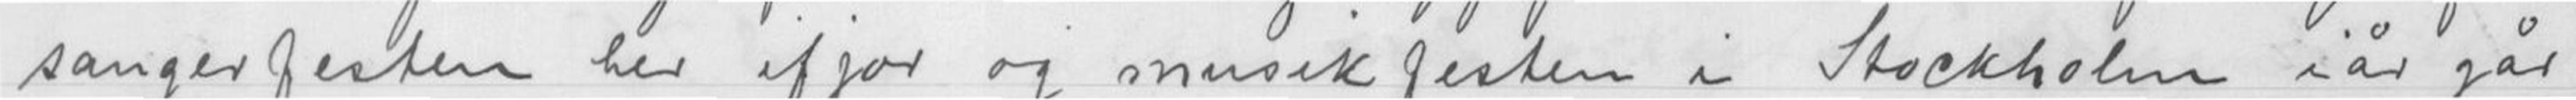sangerfesten her ifjor og musikfesten i Stockholm iår går
